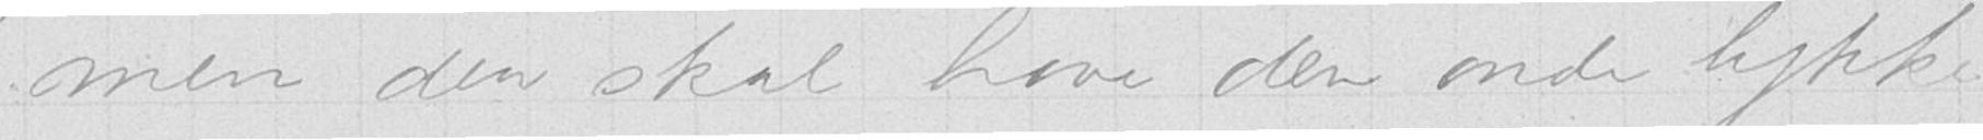"men den skal have den onde lykke
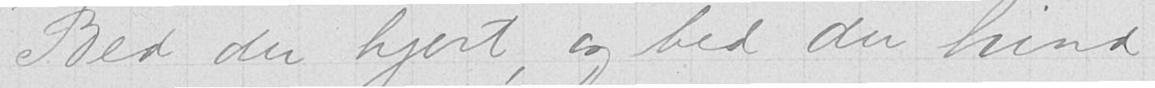"Bed du hjort, og bed du hind
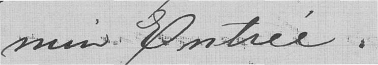min Entreé.
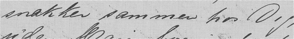snakker sammen hos Dig,
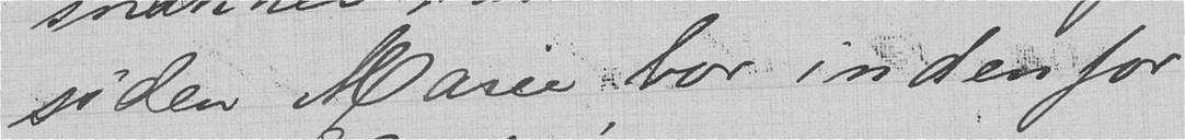siden Marie bor indenfor
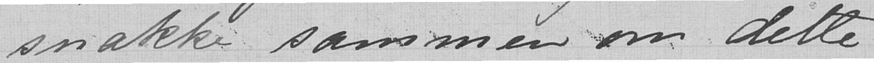snakke sammen om dette
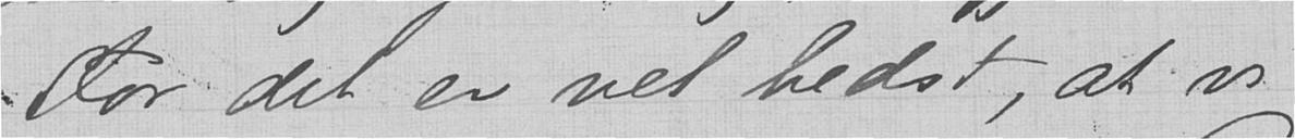For det er vel bedst, at vi
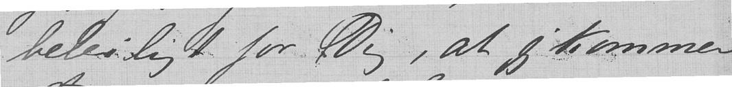beleiligt for Dig, at jeg kommer.
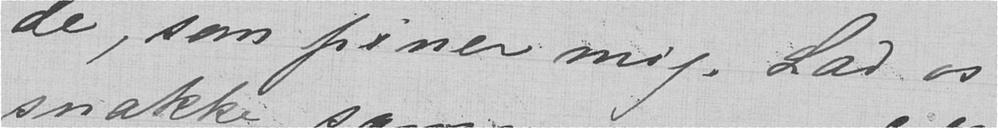de, som piner mig. Lad os
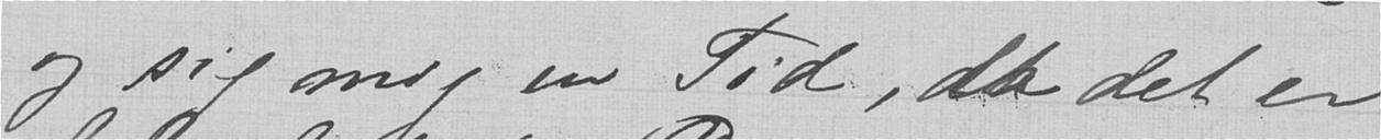og sig mig en Tid, da det er
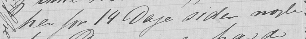her for 14 Dage siden nogle
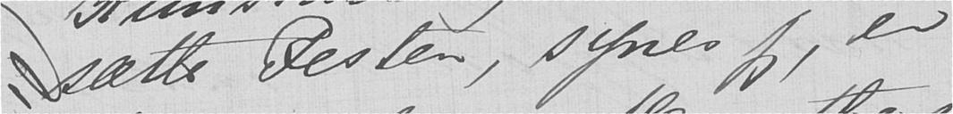sætte Festen, synes jeg, er
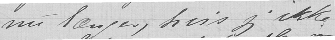nu længer, hvis jeg ikke
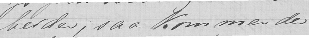beider, saa kommer der
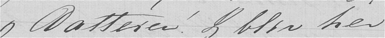og Datteren! Jeg blir her
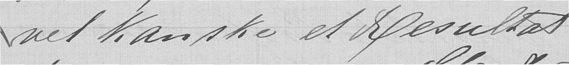vel kanske et Resultat
